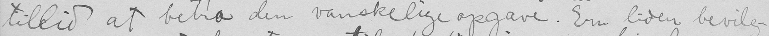tillid at betro den vanskelige opgave. En liden bevilg-
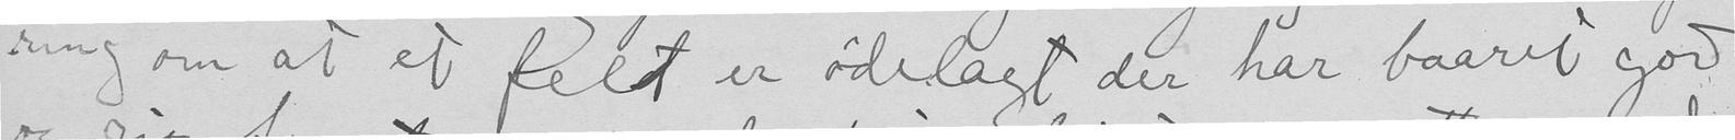ring om at et felt er ødelagt der har baaret god
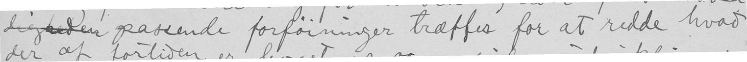pasende forføininger træffer for at redde hvad
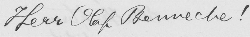Herr Olaf Benneche!
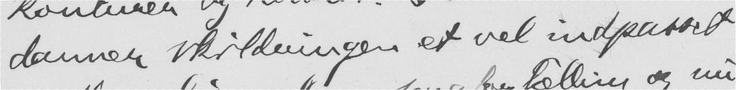danner skildringen et vel indpasset
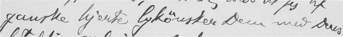ganske hjerte lykønsker Dem med Deres
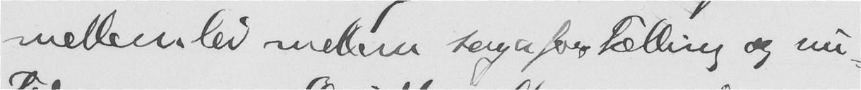mellemled mellem sagafortælling og nu-
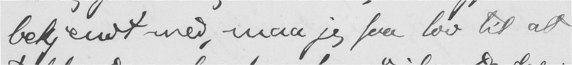bekjendt med, maa jeg faa lov til at
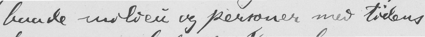baade milieu og personer med tidens
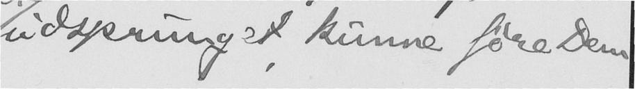udsprunget, kunne føre Dem
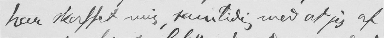har skaffet mig, samtidig med at jeg af
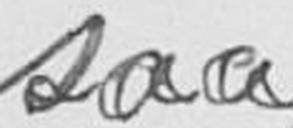saa
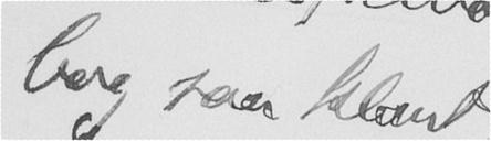bog saa klart
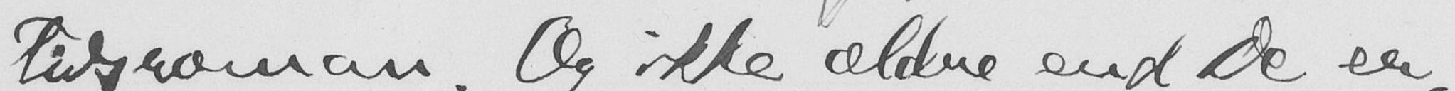tidsroman. Og ikke ældre end De er,
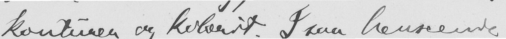konturer og kolorit. I saa henseende
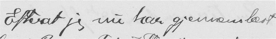Efterat jeg nu har gjennemlæst
